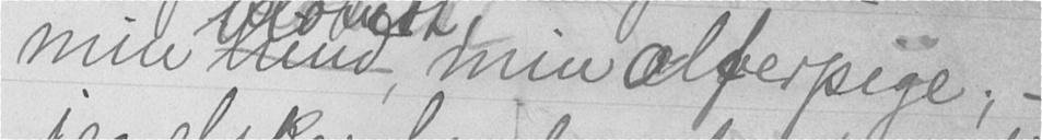min blomst, min alferpige; -
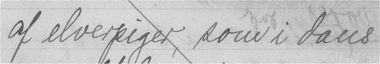af elverpiger, som i dans
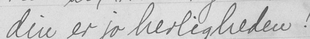din er jo herligheden!
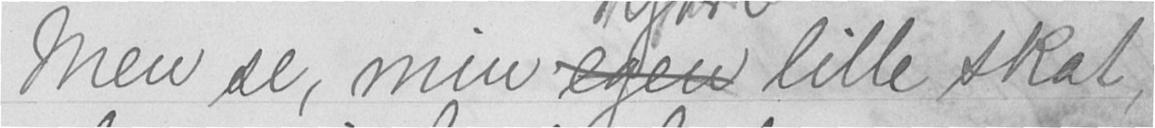Men se, min kjære lille skat,
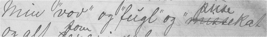Min "vov", og "fugl" og "pusekat"
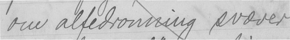om alfedronning svæver
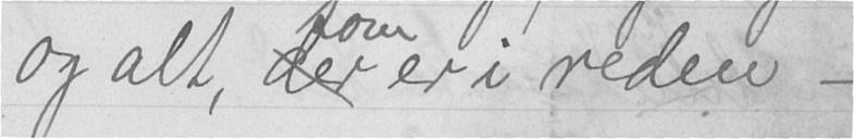og alt, som er i reden -
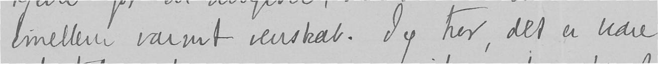imellem varmt venskab. Jeg tror, det er bedre
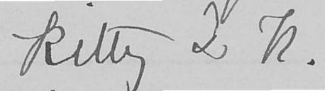Kitty L K.
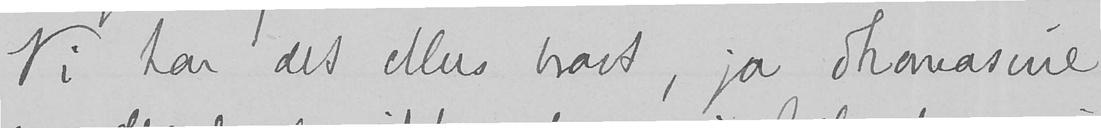Vi har det ellers bravt, ja Thomasine
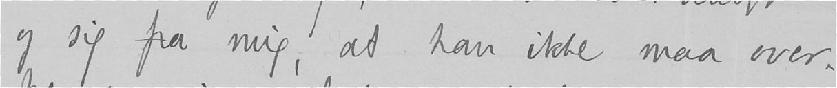og sig fra mig, at han ikke maa over-
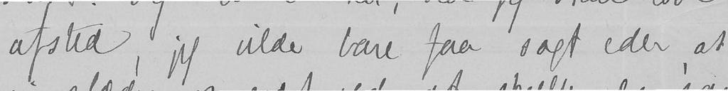afsted, jeg vilde bare faa sagt eder, at
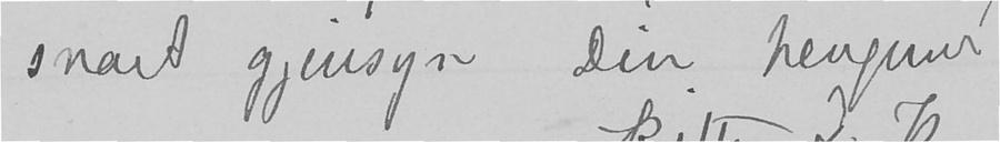snart gjensyn Din hengivne
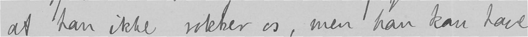at han ikke rokker os, men han kan have
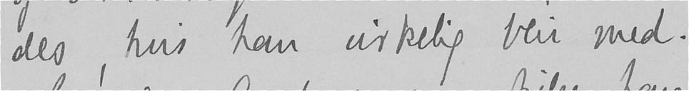des, hvis han virkelig blir med.
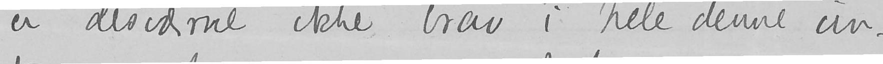er desværre ikke brav i hele denne vin-
